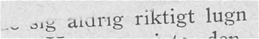e sig aidrig riktigt lugn
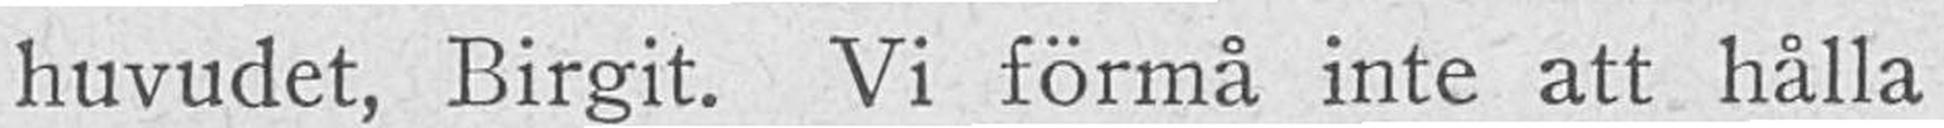huvudet, Birgit. Vi förmà inte att hålla
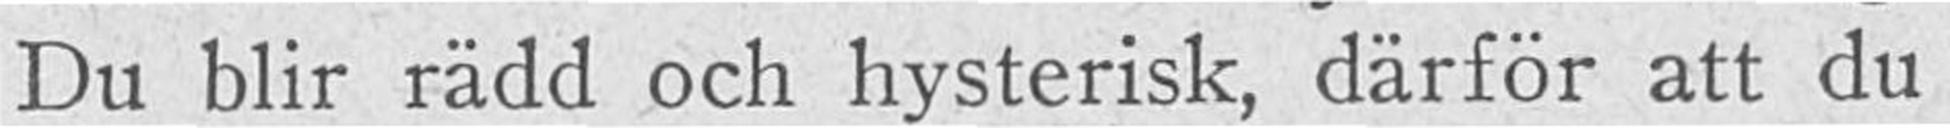Du blir rädd och hysterisk, därför att du
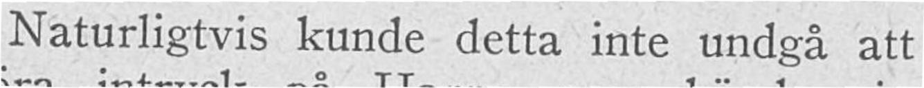Naturligtvis kunde detta inte undgå att
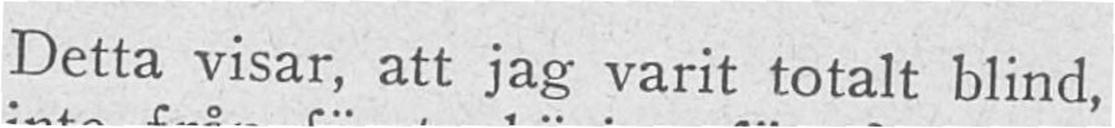- Detta visar, att jag varit totalt blind,
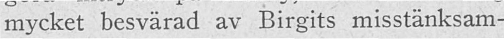mycket besvarad av Birgits misstänksam-
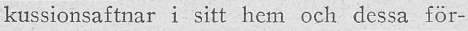kussionsaftnar i sitt hem och dessa för-
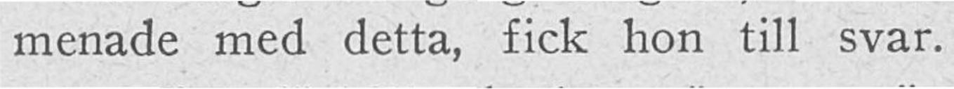menade med detta, fick hon till svar.
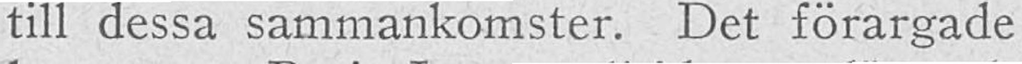till dessa sammankomster. Det förargade
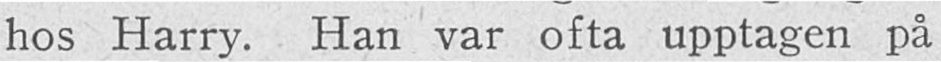hos Harry. Han var ofta upptagen på
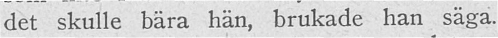det skulle bära hän, brukade han säga.
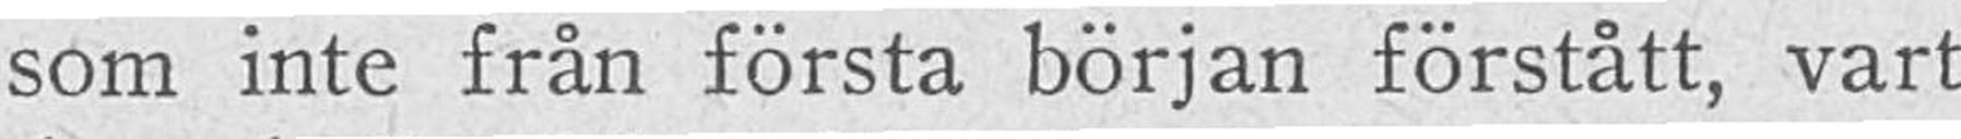som inte från första början förstätt, vart
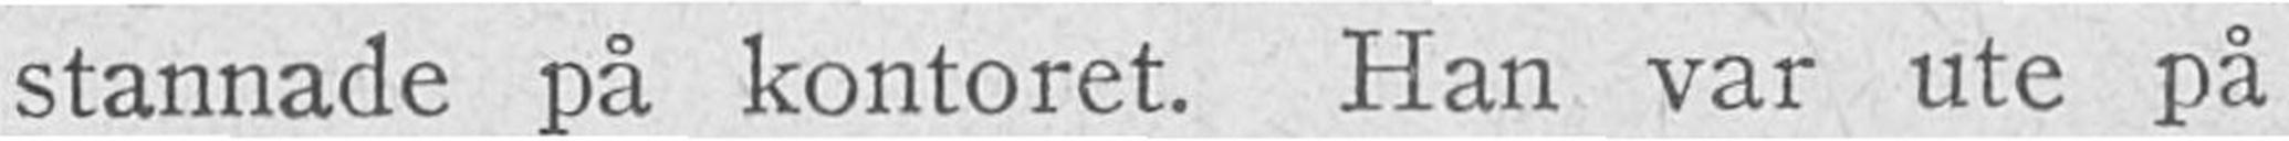stannade på kontoret. Han var ute på
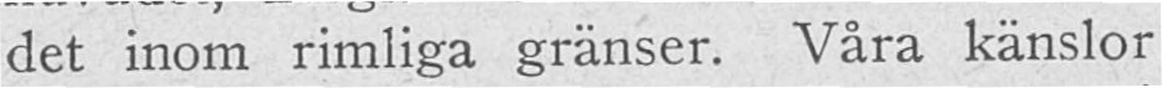det inom rimliga gränser. Våra känslor
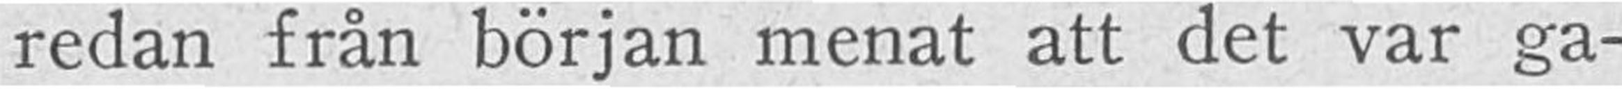redan från början menat att det var ga-
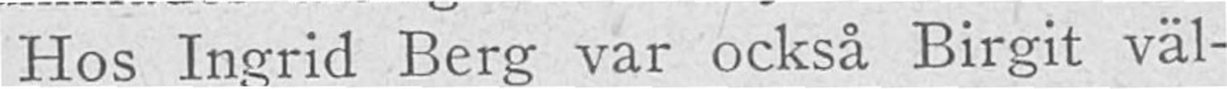Hos Ingrid Berg var också Birgit väl-
